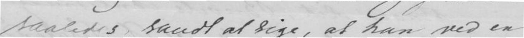saaledes sandt at sige, at han ved en
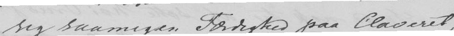seg saamegen Færdighed paa Claveret,
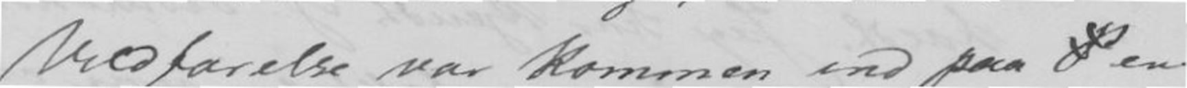Vildfarelse var kommen ind paa en
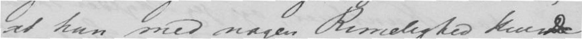at han med nogen Rimelighed kunde
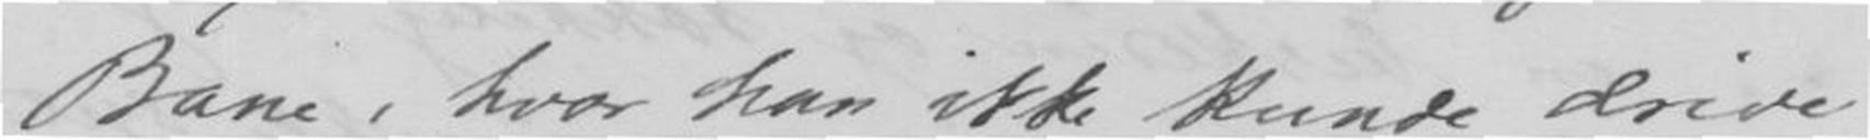Bane, hvor han ikke kunde drive
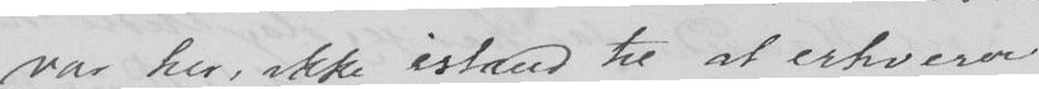var her, ikke istand til at erhverve
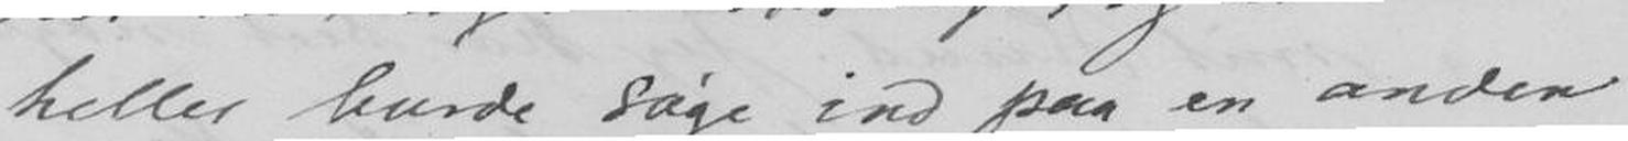heller burde søge ind paa en anden
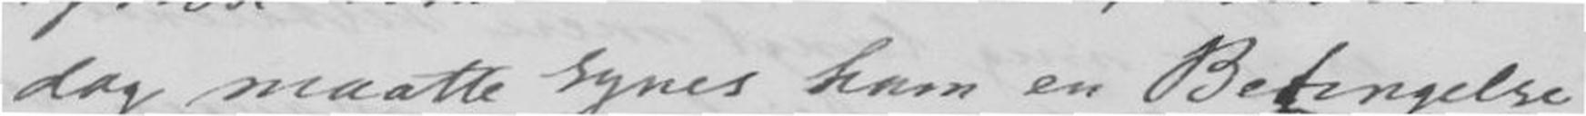dog maatte synes ham en Betingelse
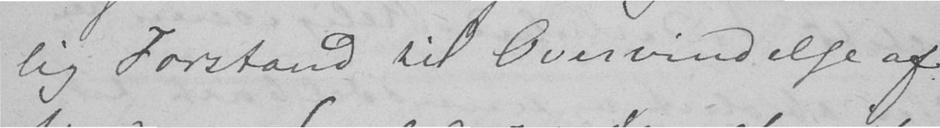lig Forstand til Overvindelse af
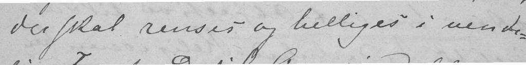der skal renses og helliges i uende-
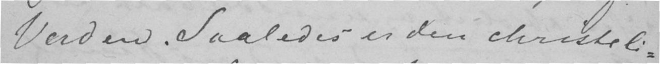Verden. Saaledes er den christeli-
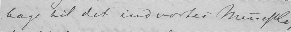bage til det indvortes Menneske,
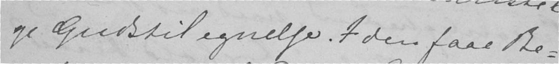ge Gudstilegnelse. I den faae Be-
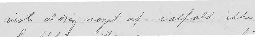vist aldrig noget af - ialfald ikke
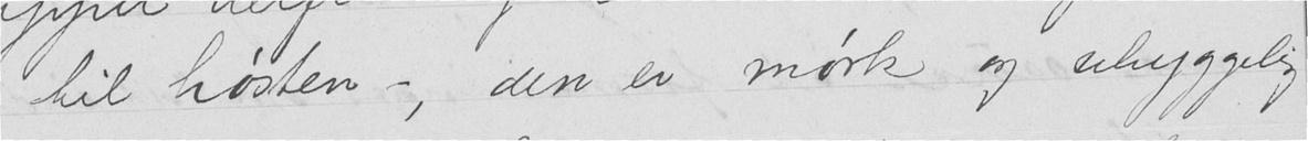til høsten -, den er mørk og uhyggelig
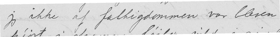jeg ikke af fattigdommen var bleven
